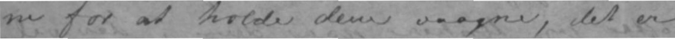ne for at holde dem vaagne, det er
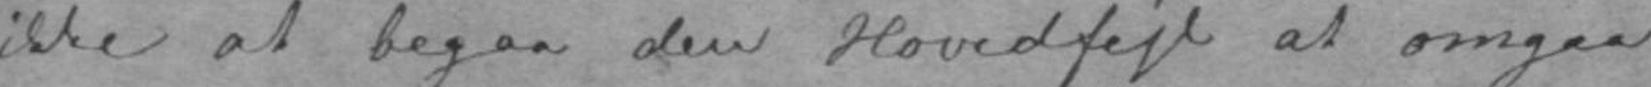ikke at begaa den Hovedfejl at omgaa
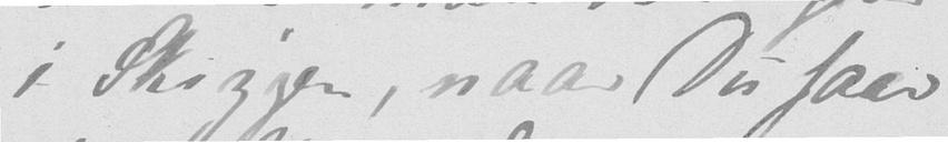i Skizzen, naar Du faar
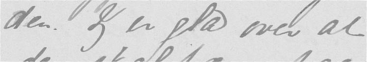den. Jeg er glad over at
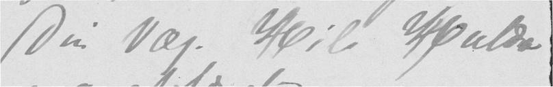Din Væg. Hils Hulda
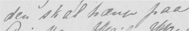den skal hænge paa
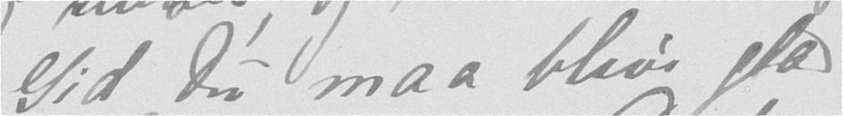Gid du maa blive glad
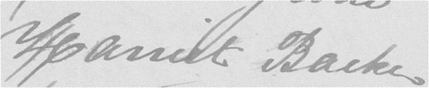Harriet Backer
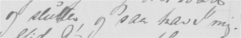og slutter, og saa har I mig.
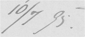10/7 95.
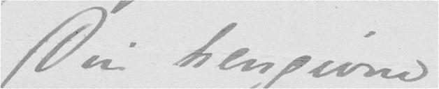Din hengivne
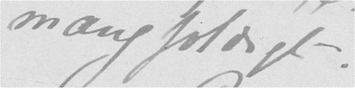mangfoldigt.
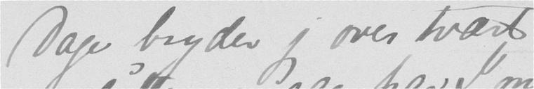Dage bryder jeg over tvært
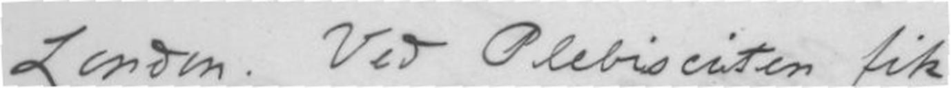London. Ved Plebisciten fik
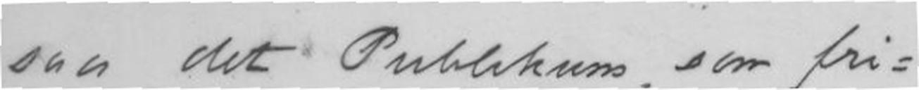saa det Publikum, som fri-
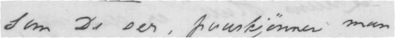Som De ser, paaskjønner man
